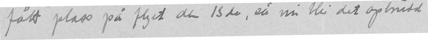fått plass på flyet den 13de, så nu blir det ogbrudd
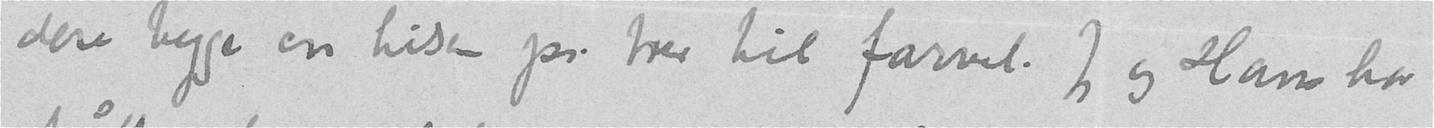dere begge en hilsen pr. brev til farvel. Jeg og Hans har
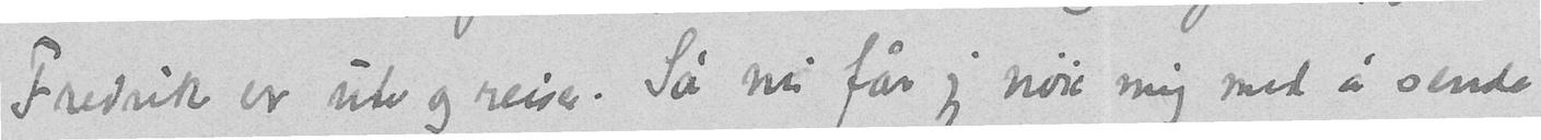Fredrik er ute og reiser. Så nu får jeg nøie mig med å sende
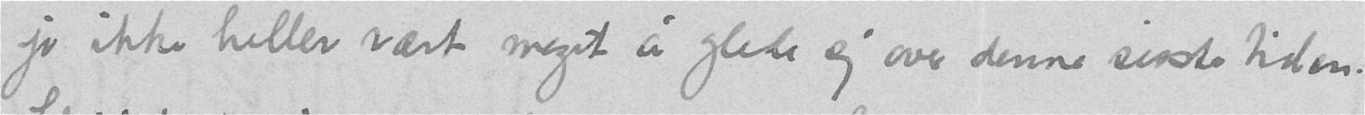jo ikke heller vært meget å glede sig over denne sisste tiden.
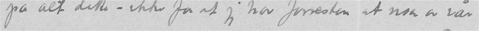på alt dette – ikke for at jeg tror forresten at noen av vår
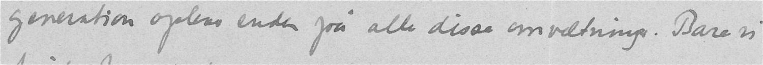generation oplever enden pa alle disse omveltninger. Bare vi
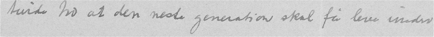turde tro at den neste generation skal få leve under
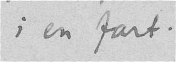i en fart.
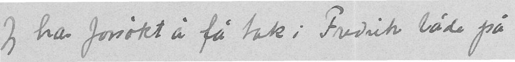Jeg har forsøkt å få tak i Fredrik både på
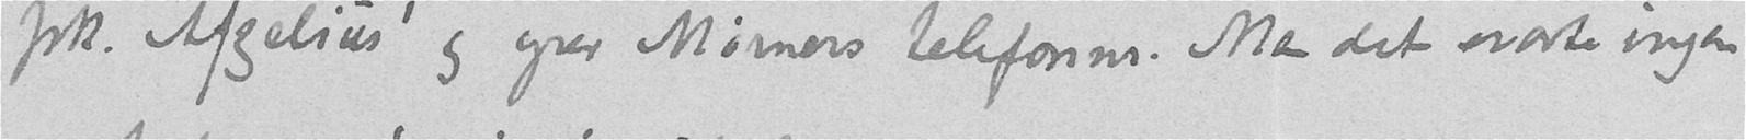frk. Afzelius' og grev Mørners telefonnr. Men det svarte ingen
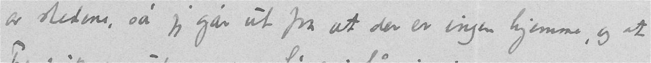av stedene, så jeg går ut fra at der er ingen hjemme, og at
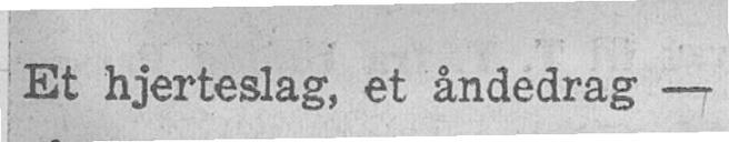Et hjerteslag, et åndedrag —
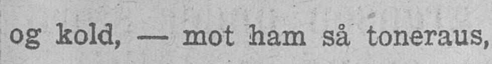og kold, — mot ham så toneraus,
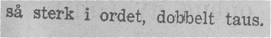så sterk i ordet, dobbelt taus.
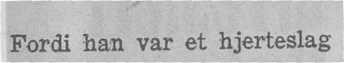Fordi han var et hjerteslag
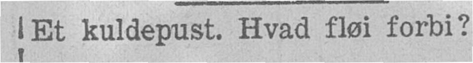Et kuldepust. Hvad fløi forbi?
